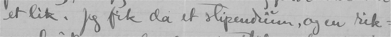et lik. Jeg fik da et stipendnium, og en rik-
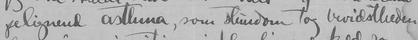pelignend asthma, som stundom tog bevidstheden
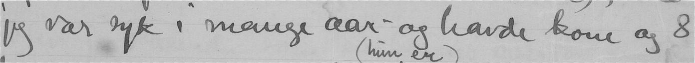jeg var syk i mange aar - og havde kone og 8
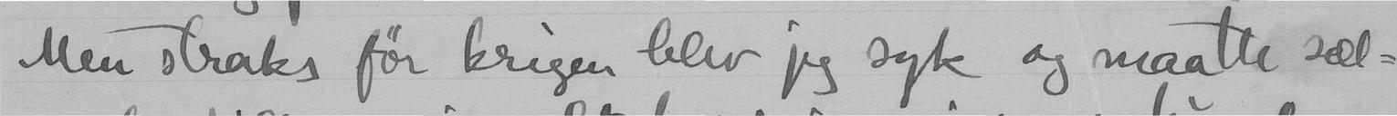Men straks før krigen blev jeg syk og maatte sæl-
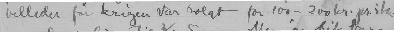billeder før krigen var solgt for 100-200 kr. pr. stk.
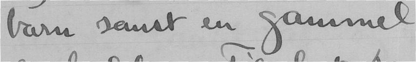barn samt en gammel
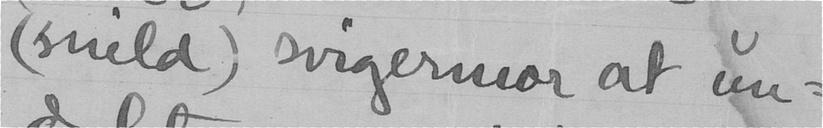(snild) svigermor at un-
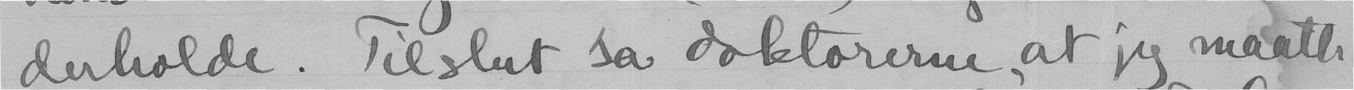derholde. Tilslut sa doktorerne, at jeg maatte
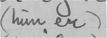(hun er)
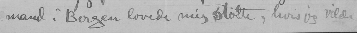mand i Bergen lovede mig støtte, hvis jeg vilde
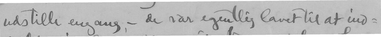udstille engang, - de var egentlig lavet til at ind-
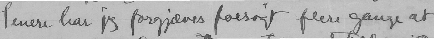Senere har jeg forgjæves forsøgt flere gange at
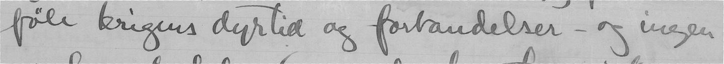føle krigens dyrtid og forbandelser - og ingen
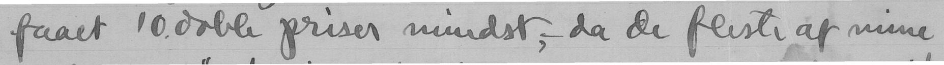faaet 10 doble priser mindst, - da de fleste af mine
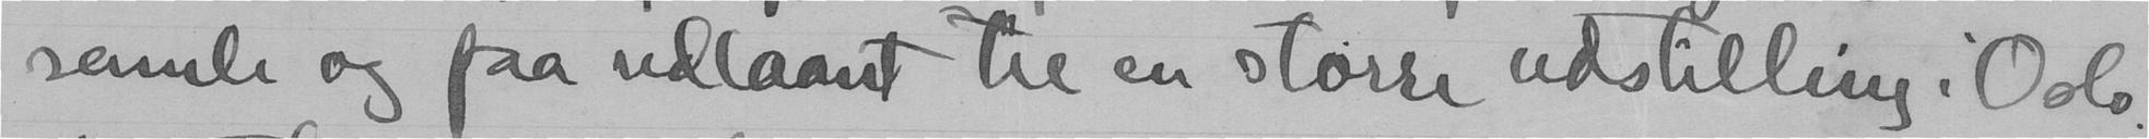samle og faa udlaant til en større udstilling i Oslo.
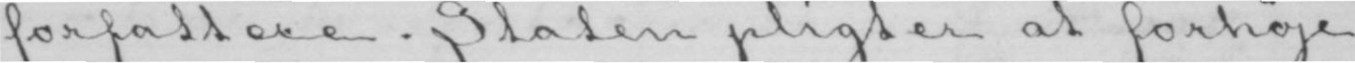forfattere. Staten pligter at forhøje
